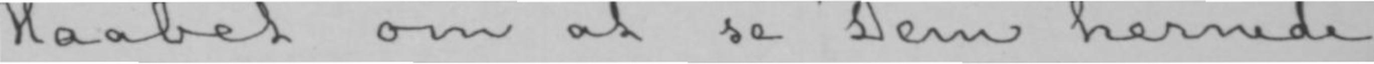Haabet om at se Dem hernede
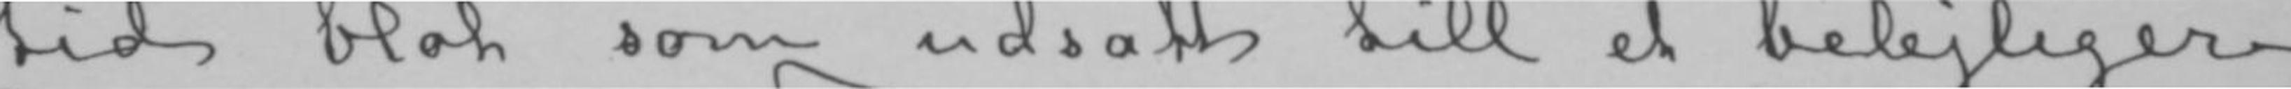tid blot som udsatt till et belejligere
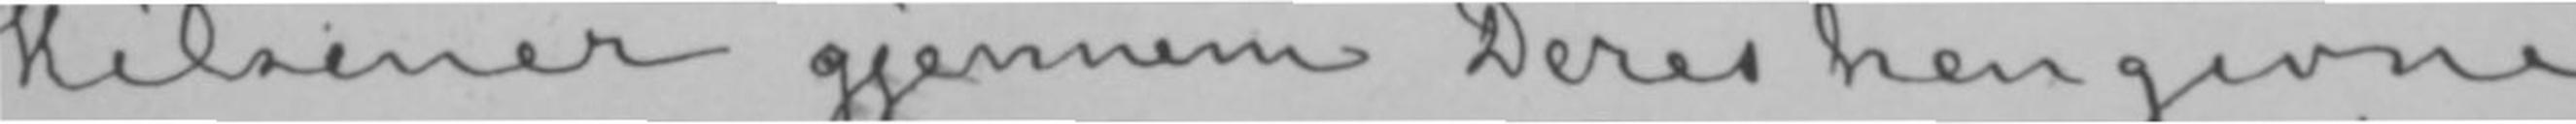Hilsener gjennem Deres hengivne
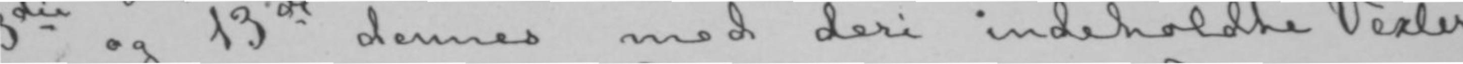3die og 13de dennes med deri indeholdte Vexler
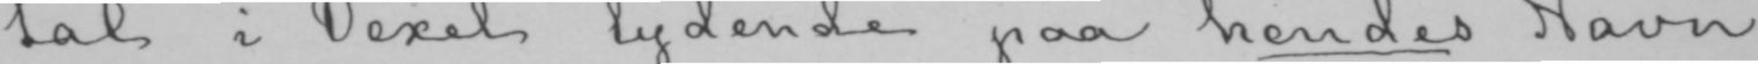tal i Vexel lydende paa hendes Navn
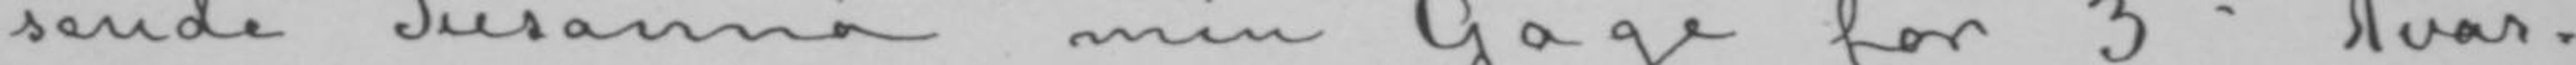sende Susanna min Gage for 3die Kvar-
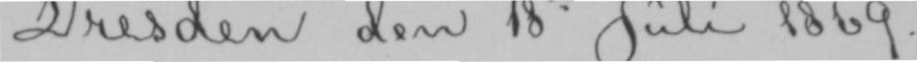Dresden den 18de Juli 1869.
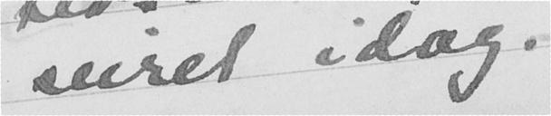seiret idag.
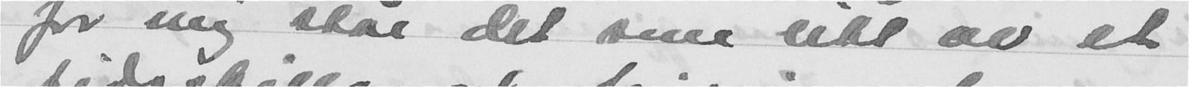for mig står det som litt av et
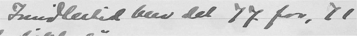Imidlertid blev det 77 for, 71
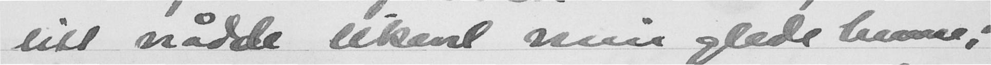litt nådde likevel min glede henne;
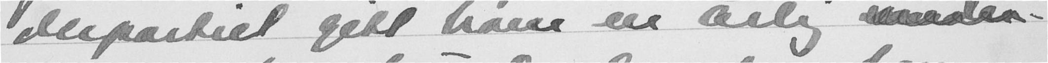derpartiet gitt ham en årlig
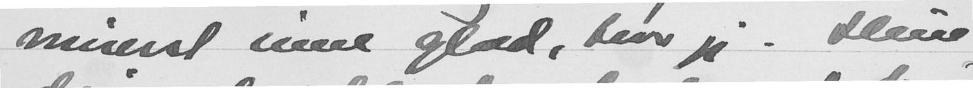innerst inne glad, tror jeg. Hun
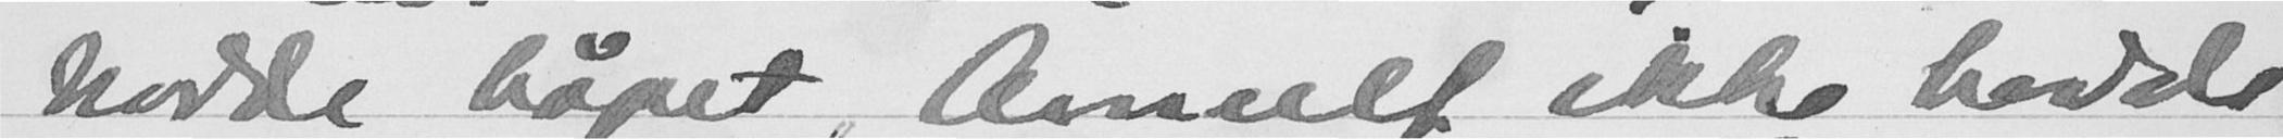hadde håpet, Arnulf ikke havde
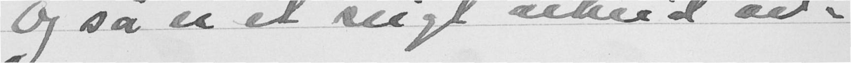Og så er et seigt arbeid av-
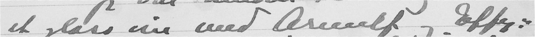et glass vin med Arnulf og Effy.
